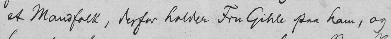et Mandfolk, derfor holder Fru Gihle paa ham, og
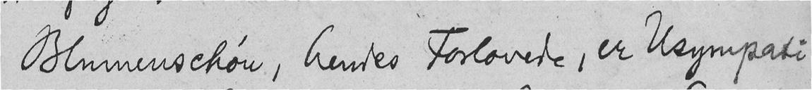Blumenschøn, hendes Forlovede, er Usympati
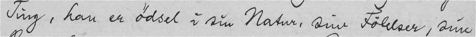Ting, han er ødsel i sin Natur, sine Følelser, sine
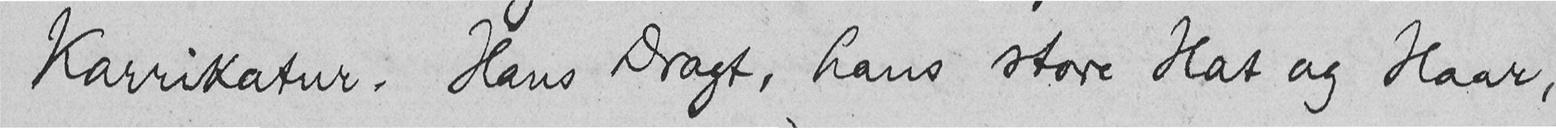Karrikatur. Hans Dragt, hans store Hat og Haar,
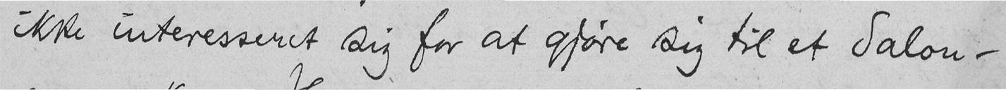ikke interesseret sig for at gjøre sig til et Salon-
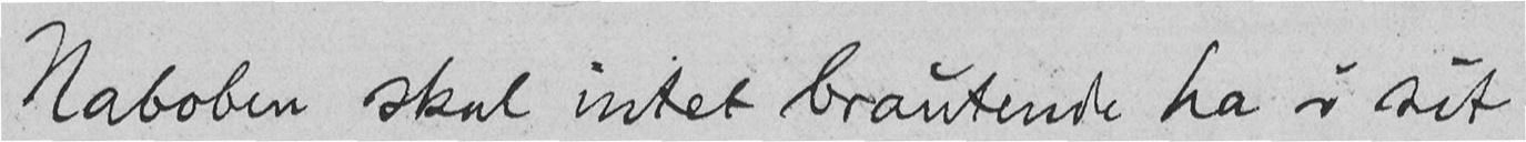Naboben skal intet brautende ha i sit
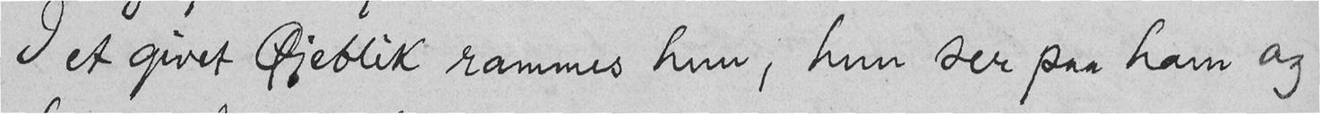I et givet Øjeblik rammes hun, hun ser paa ham og
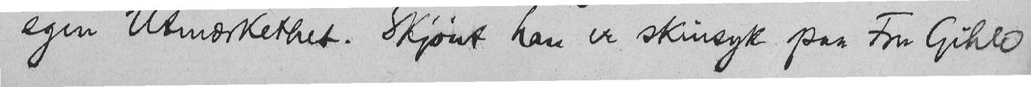egen Utmærkethet. Skjønt han er skinsyk paa Fru Gihle
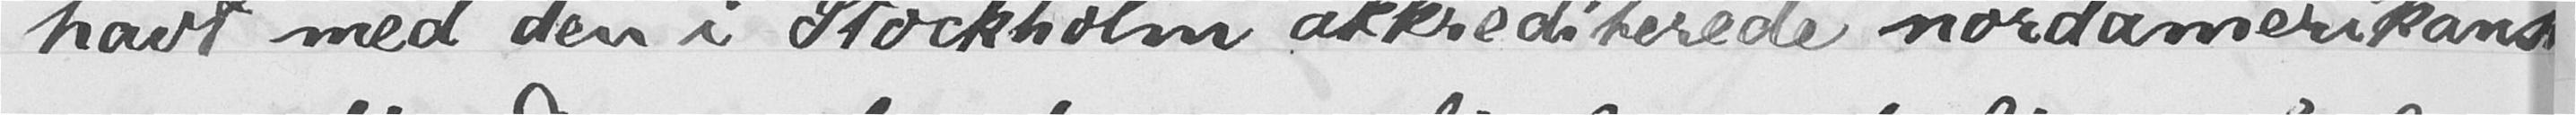havt med den i Stockholm akkrediterede nordamerikanske
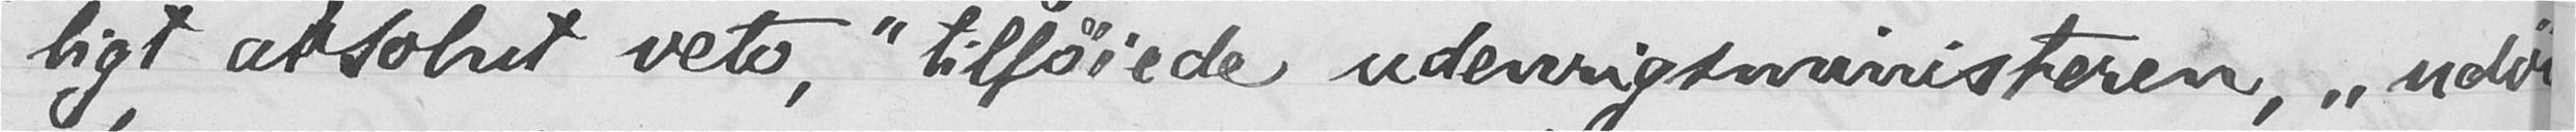ligt absolut veto," tilføiede udenrigsministeren, "udøves
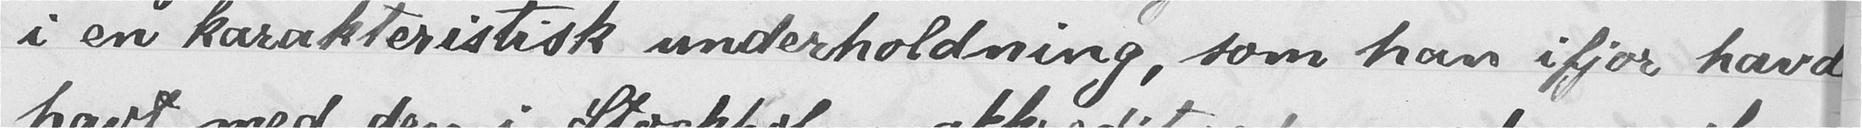i en karakteristisk underholdning, som han ifjor havde
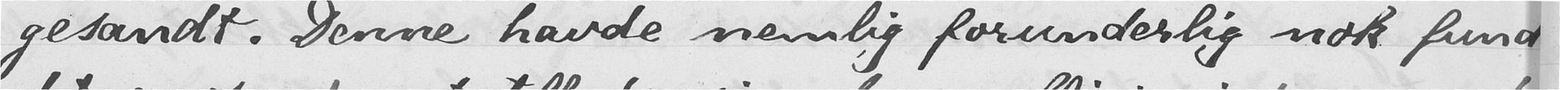gesandt. Denne havde nemlig forunderlig nok fundet
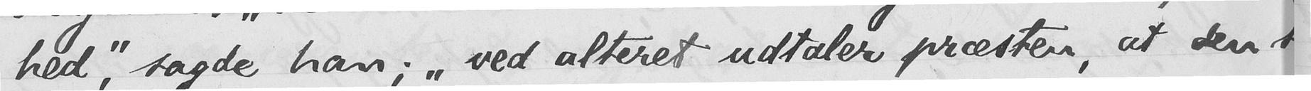hed", sagde han; "ved alteret udtaler præsten, at den slut-
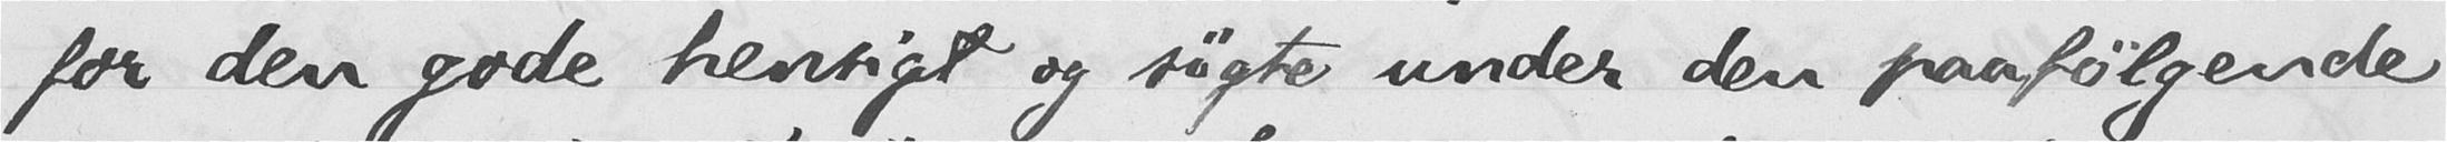for den gode hensigt og søgte under den paafølgende
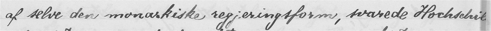af selve den monarkiske regjeringsform, svarede Hochschild:
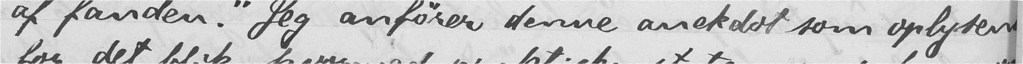af fanden." Jeg anfører denne anekdot som oplysende
